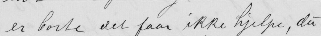er borte det faar ikke hjælpe, du
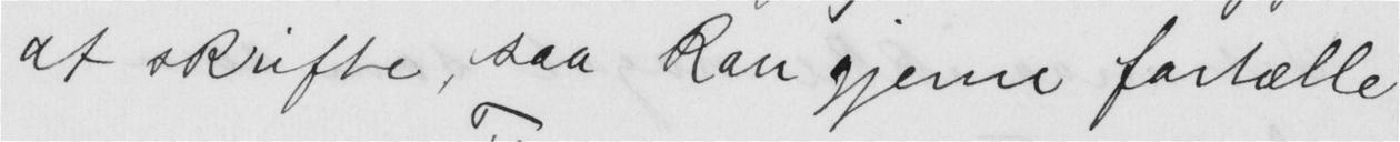at skrifte, saa kan gjerne fortælle
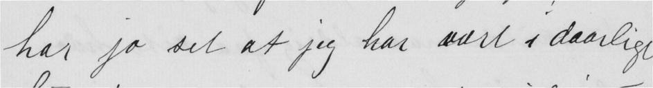har jo det at jeg har vært i daarligt
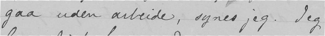gaa uden arbeide, synes jeg. Jeg
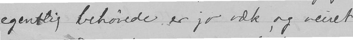egentlig behøvede er jo væk, og veiret
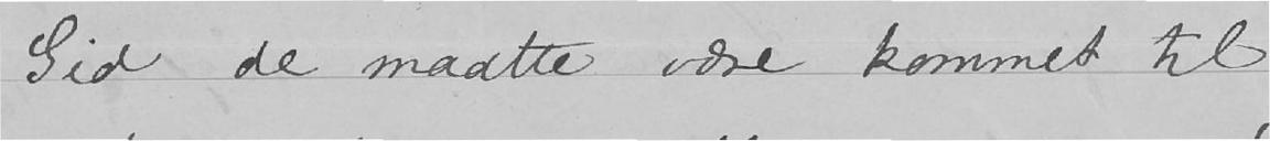Gid de maatte være kommet til
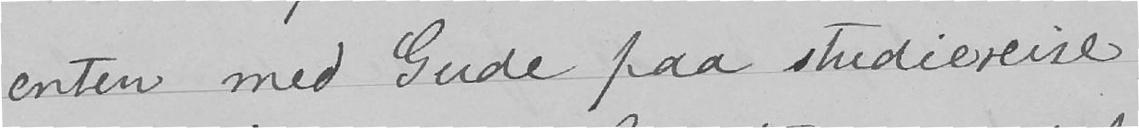enten med Gude paa studiereise
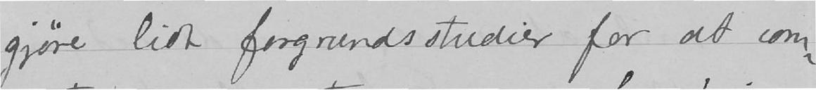gjøre lidt forgrundsstudier for at com-
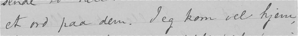et ord paa dem. Jeg kom vel hjem,
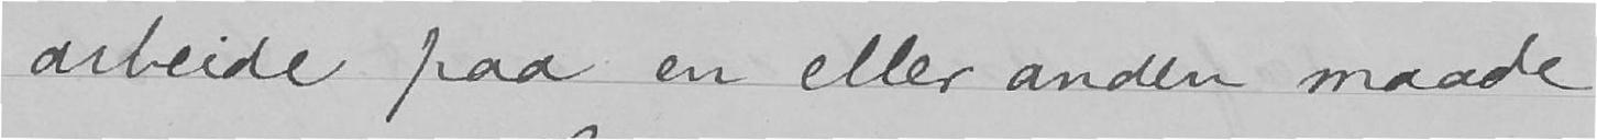arbeide paa en eller anden maade
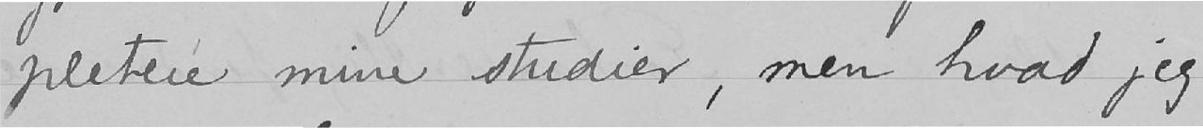pletere mine studier, men hvad jeg
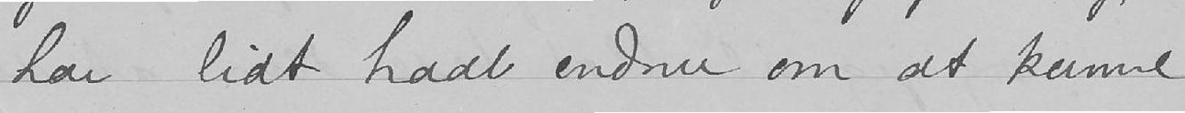har lidt haab endnu om at kunne
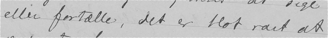eller fortælle, det er blot rart at
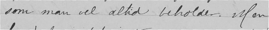som man vel altid beholder. Men
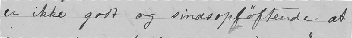er ikke godt og sindsopløftende at
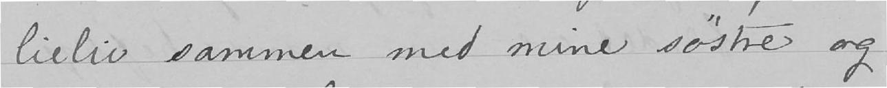lieliv sammen med mine søstre og
